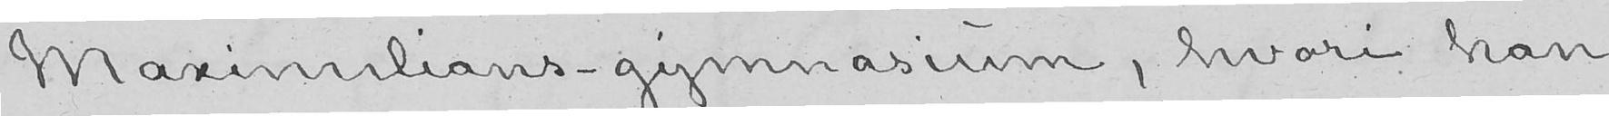Maximilians-gymnasium, hvori han
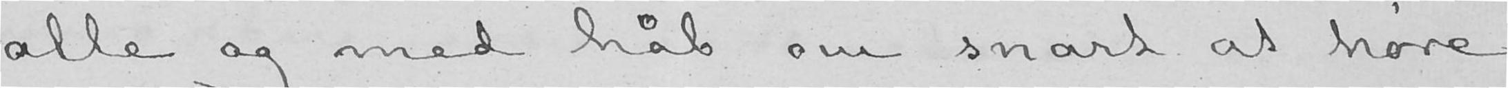alle og med håb om snart at høre
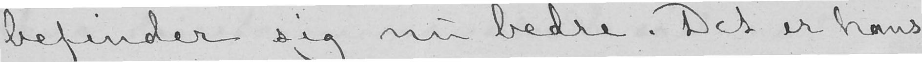befinder sig nu bedre. Det er hans
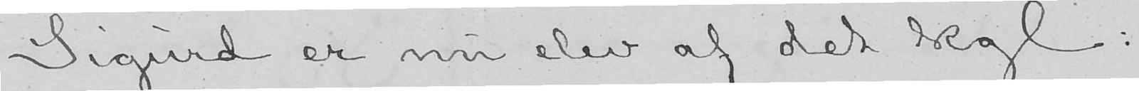Sigurd er nu elev af det kgl:
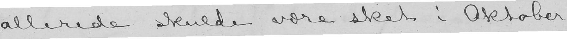allerede skulde være sket i Oktober
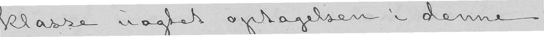klasse uagtet optagelsen i denne
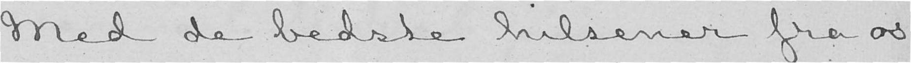Med de bedste hilsener fra os
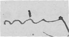mig
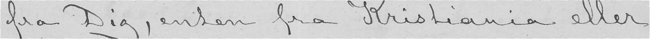fra Dig, enten fra Kristiania eller
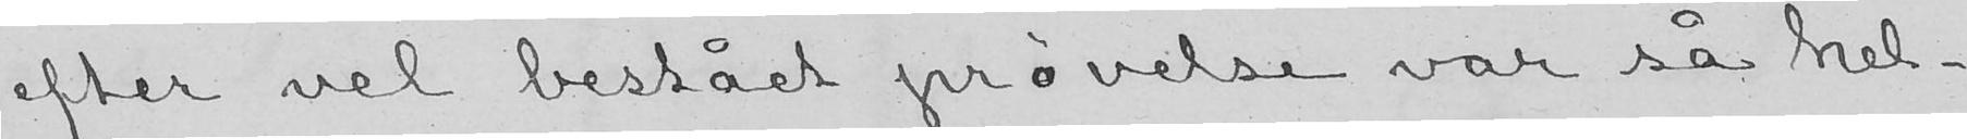efter vel bestået prøvelse var så hel-
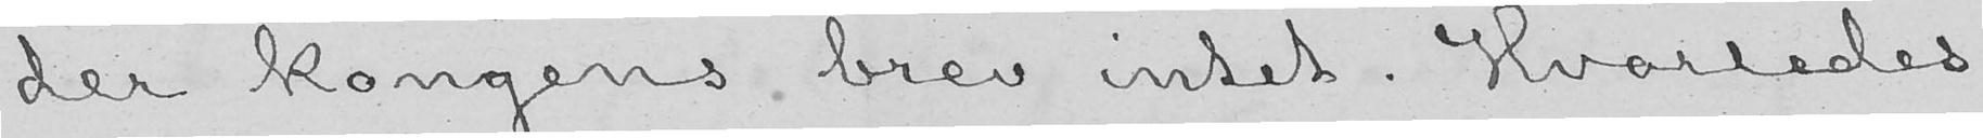der kongens brev intet. Hvorledes
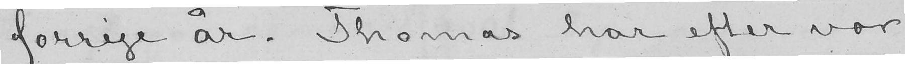forrige år. Thomas har efter vor
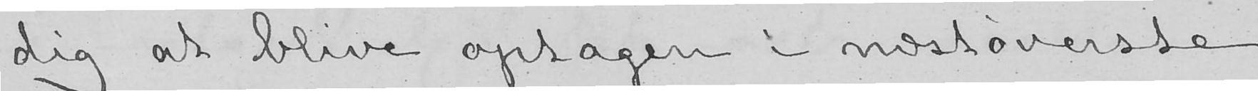dig at blive optagen i næstøverste
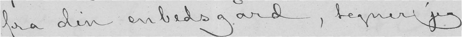fra din embedsgård, tegner jeg
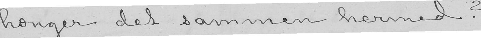hænger det sammen hermed?
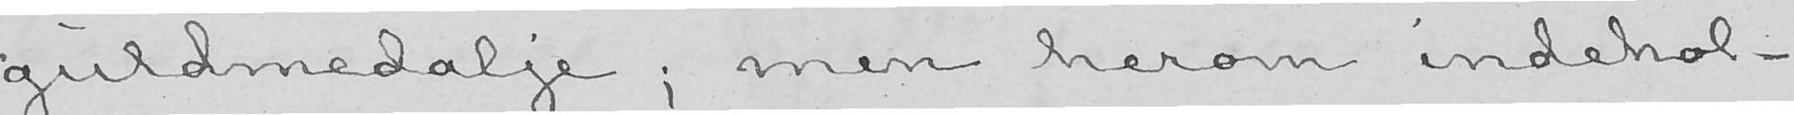guldmedalje; men herom indehol-
